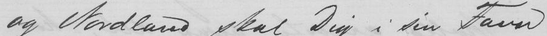og Nordland skal Dig i sin Favn
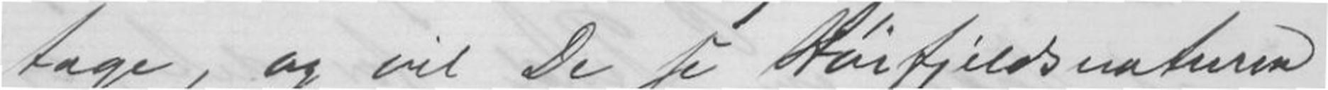tage, og vil De se Høifjeldsnaturen,
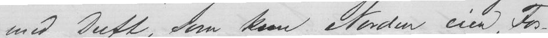med Duft, som kun Norden eier, Fos-
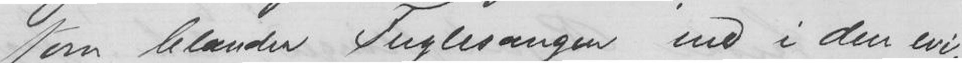som blander Fuglesangen med i den evi-
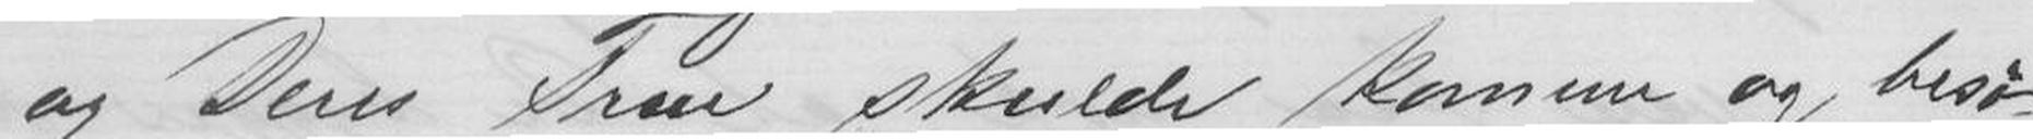og Deres Frue skulde komme og besø-
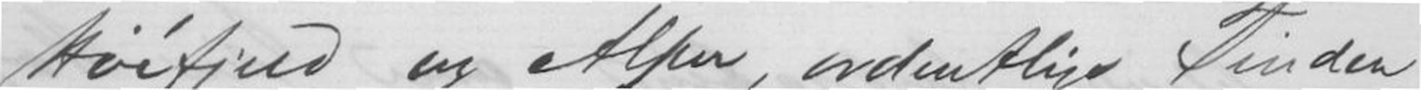Hvitfjeld og Alper, ordentlige Tinden
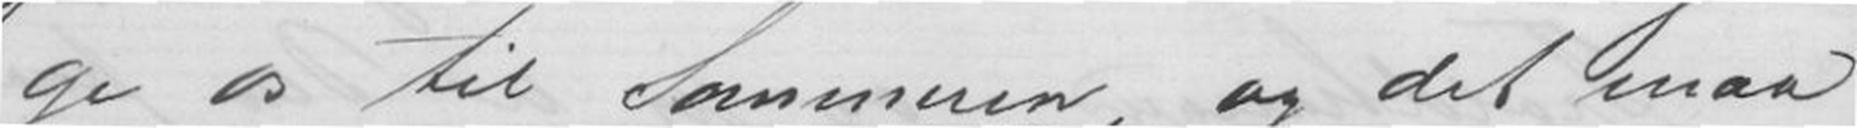ge os til Sommeren, og det maa
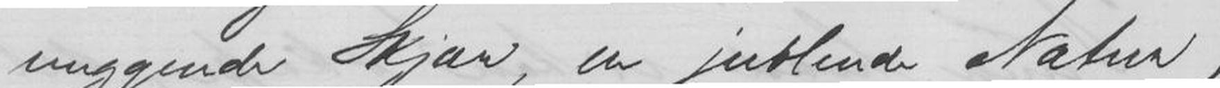vuggende Skjær, en jublende Natur,
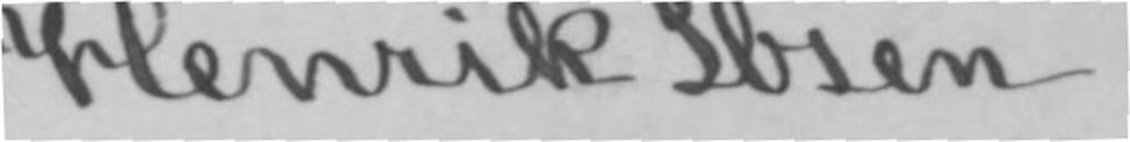Henrik Ibsen.
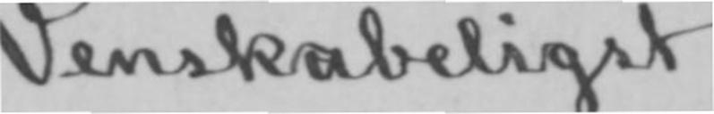Venskabeligst
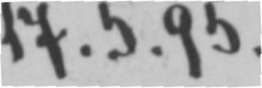17.5.95.
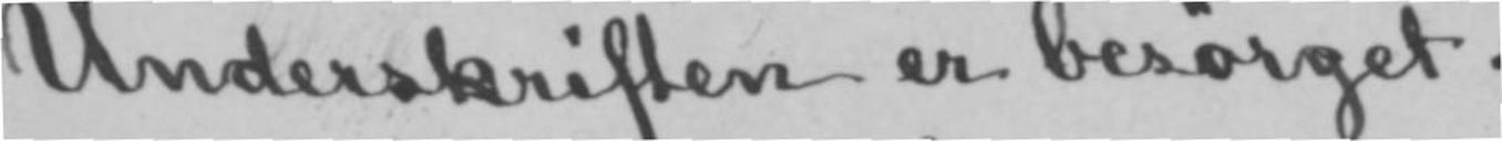Underskriften er besørget.
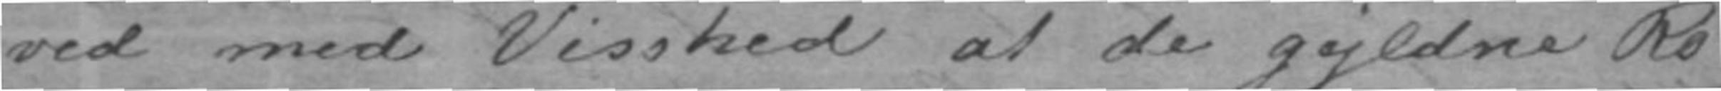ved med Visshed at de gyldne Ro-
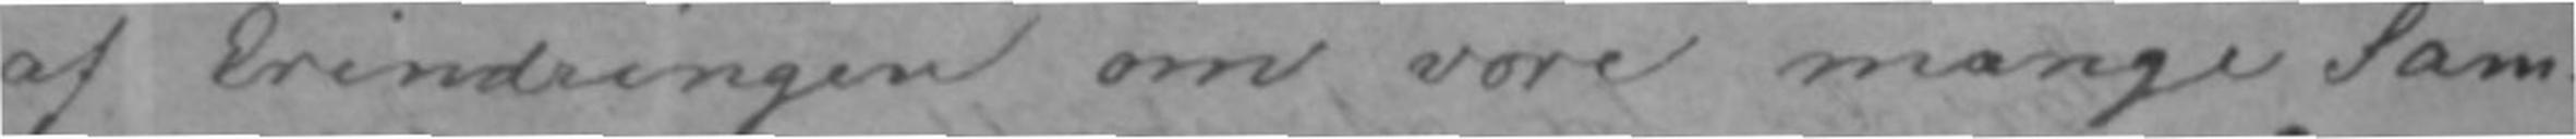af Erindringen om vore mange Sam-
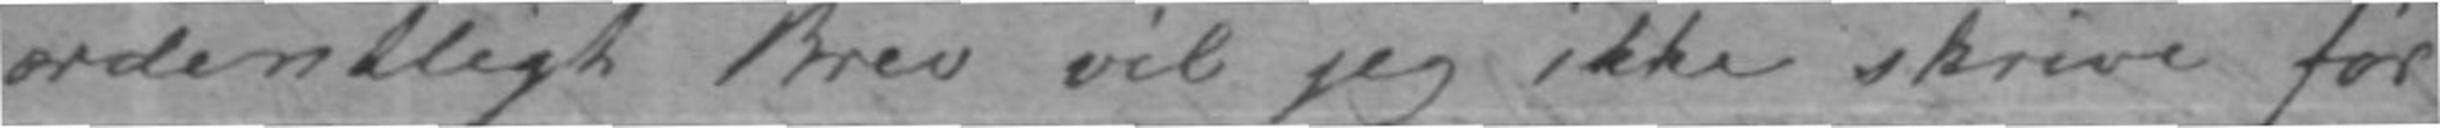ordentligt Brev vil jeg ikke skrive før
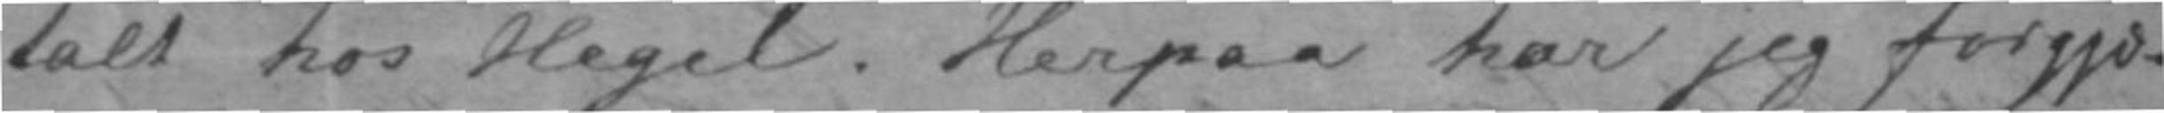talt hos Hegel. Herpaa har jeg forgjæ-
